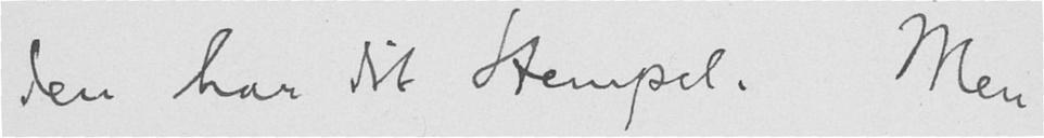den har dit Stempel. Men
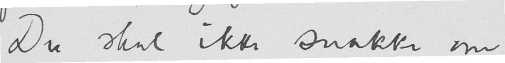Du skal ikke snakke om
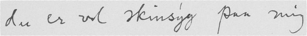du er vel skinsyg paa mig
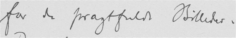for de pragtfulde Billeder.
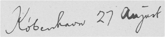København 27 August
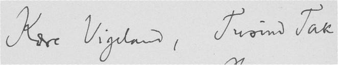Kære Vigeland, Tusind Tak
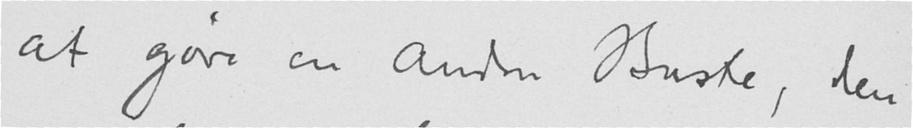at gøre en anden Buste, den
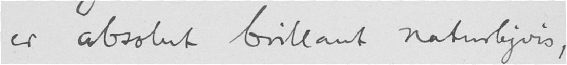er absolut brillant naturligvis,
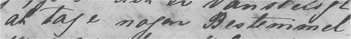at tage nogen Bestemmel-
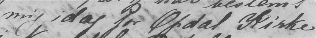mig idag for Opdal Kirke
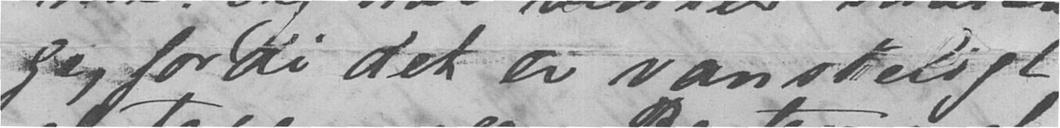ge, fordi det er vanskeligt
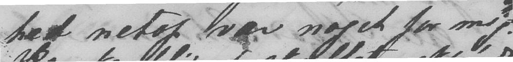hed netop var noget for mig.
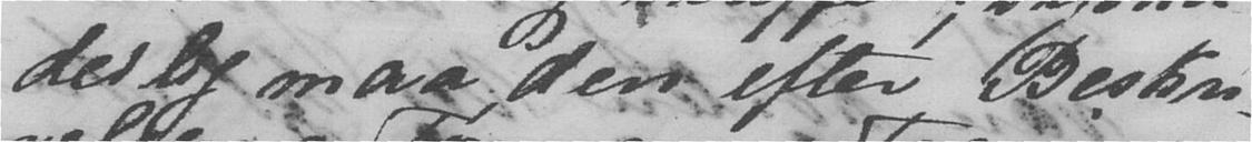deilig maa den efter Beskri-
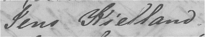Jens Kielland.
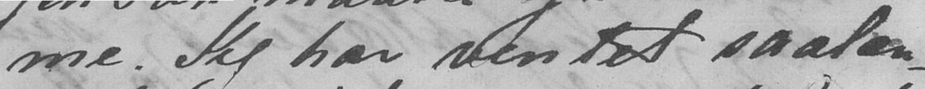me. Jeg har ventet saalen-
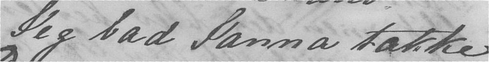Jeg bad Janna takke
